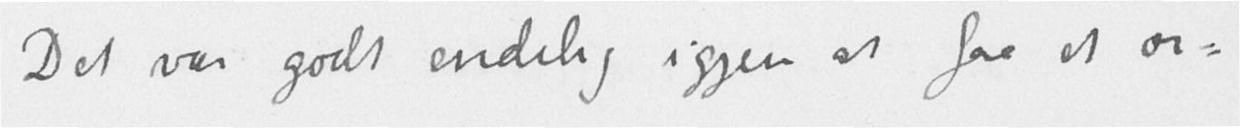Det var godt endelig igjen at faa et or-
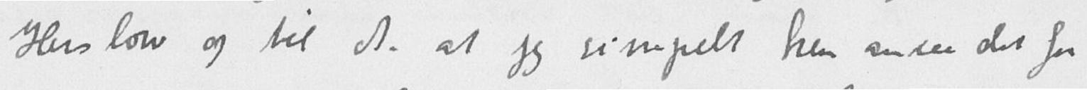Herslow og til A. at jeg simpelt hen anser det for
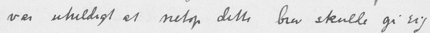var uheldigt at netop dette brev skulde gi sig
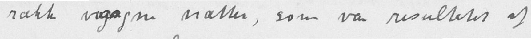række vaagne nætter, som var resultatet af
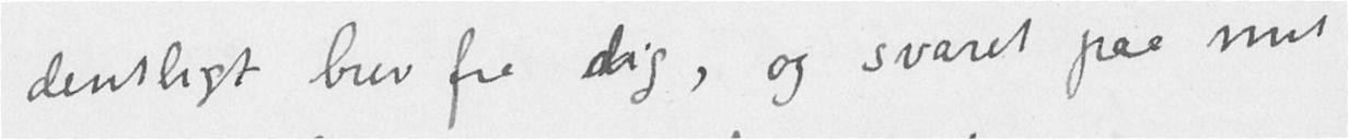dentligt brev fra dig, og svaret paa mit
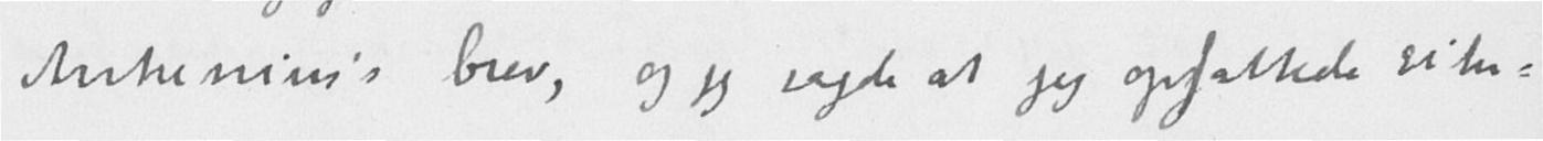Arrhenius's brev, og jeg sagde at jeg opfattede situ-
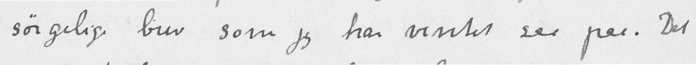sørgelige brev som jeg har ventet saa paa. Det
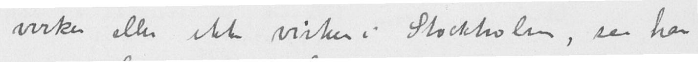virker eller ikke virker i Stockholm, saa har
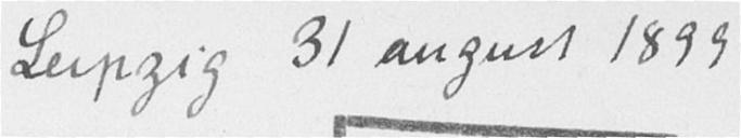Leipzig 31 august 1899
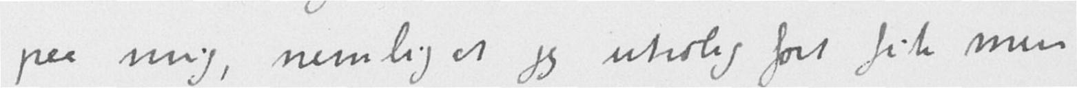paa mig, nemlig at jeg utrolig fort fik min
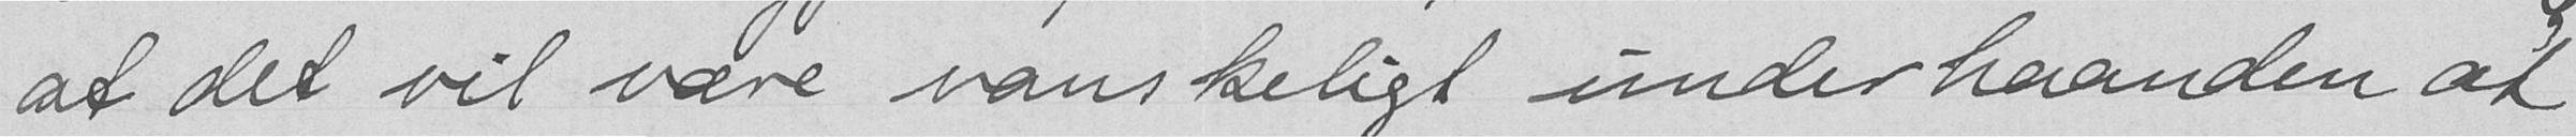at det vil være vanskeligt underhaanden at
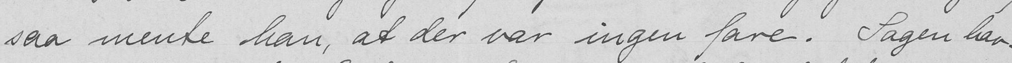saa mente han, at der var ingen fare. Sagen hav-
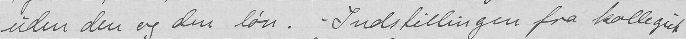uden den og den løn. Indstillingen fra kollegiet
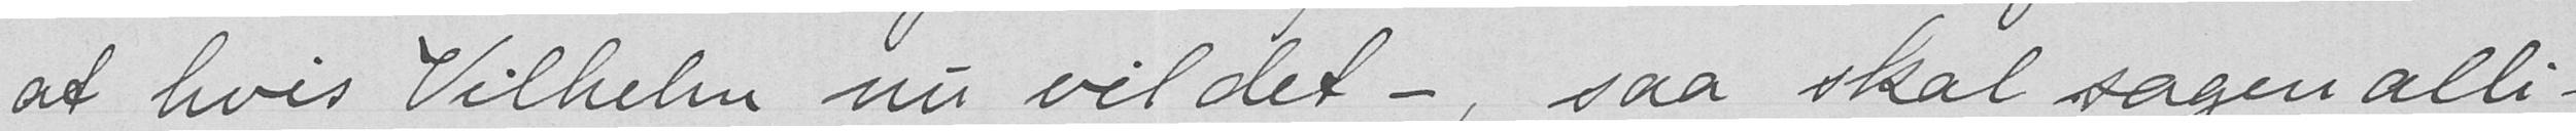at hvis Vilhelm nu vil det -, saa skal sagen alli-
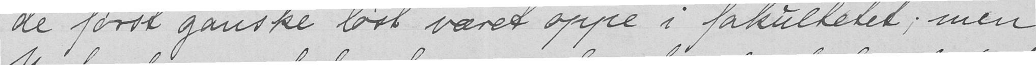de først ganske løst været oppe i fakultetet; men
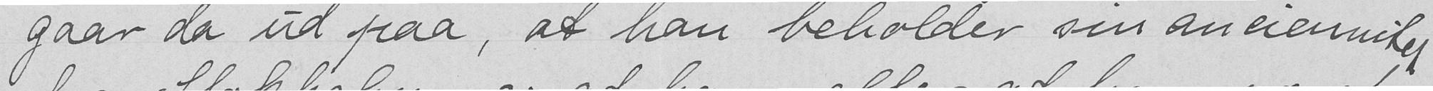gaar da ud paa, at han beholder sin anciennitet
